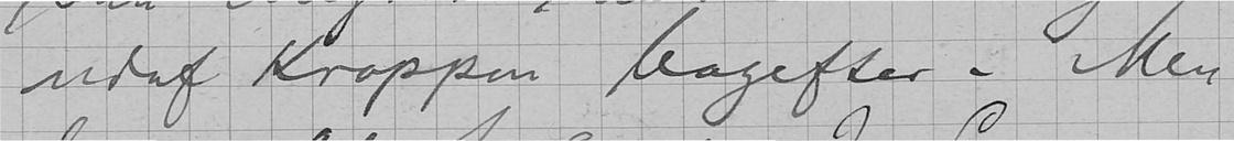udaf Kroppen bagefter. Men
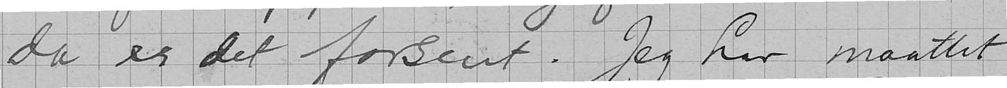da er det forsent. Jeg har maattet
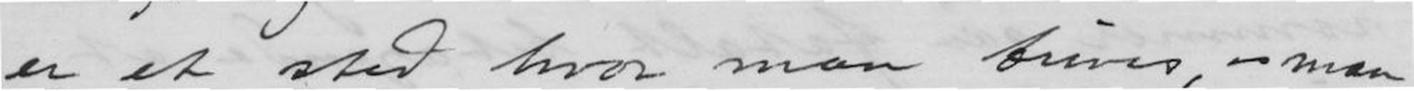er et sted hvor man trives, - man
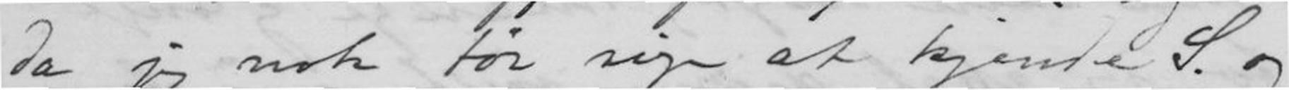da jeg nok tør sige at kjende S. og
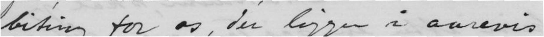biting for os, der ligger i aarevis
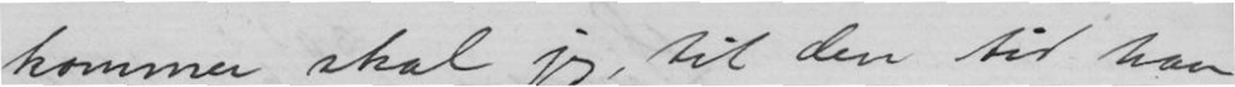kommer skal jeg til den tid han
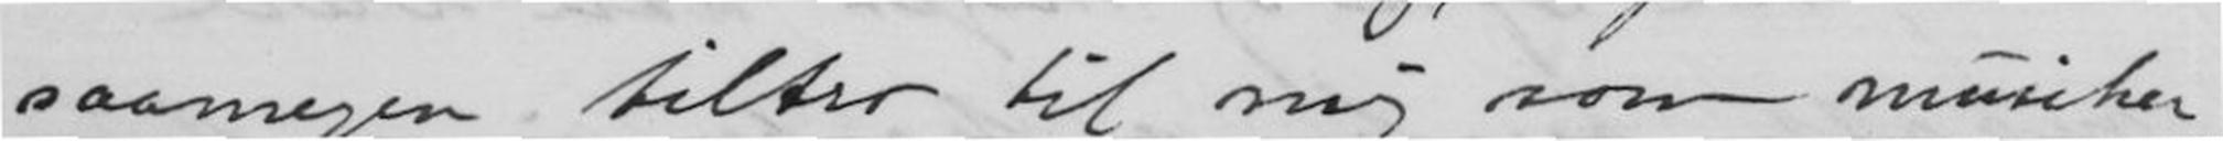saamegen tiltro til mig som musiker,
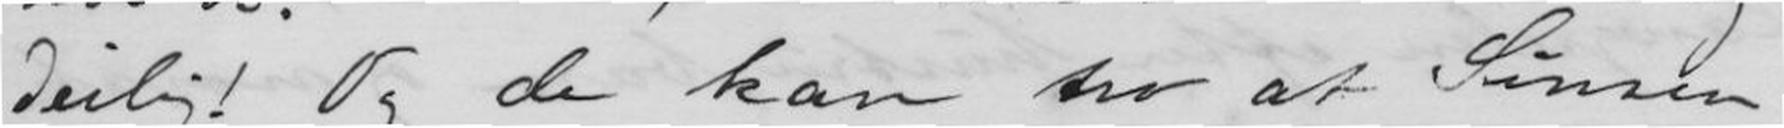deilig! Og de kan tro at Sinsen
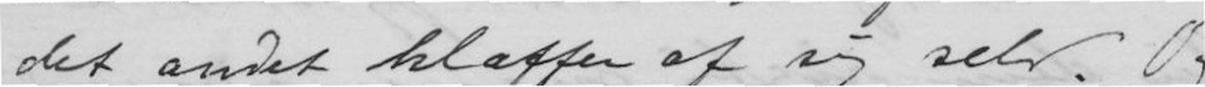det andet klaffer af sig selv. Og
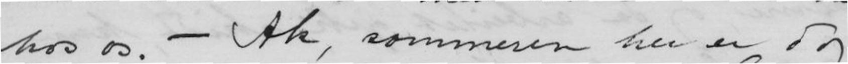hos os. - Ak, sommeren her er dog
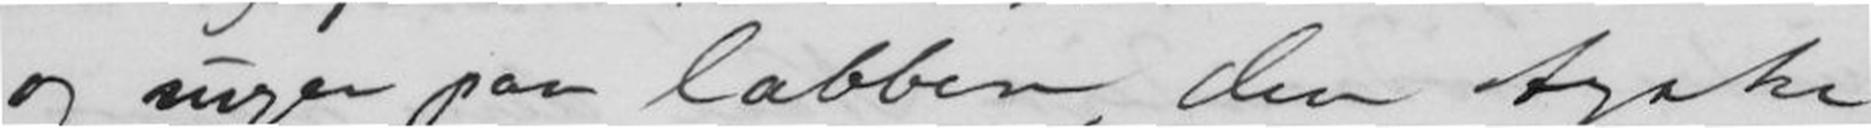og suger paa labben, den tyske
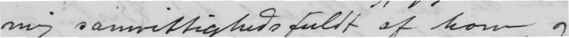tage mig samvittighedsfuldt af ham, og
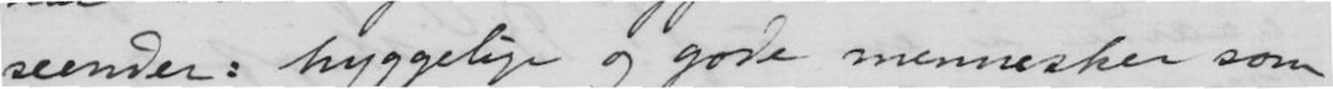seende: hyggelige og gode mennesker som
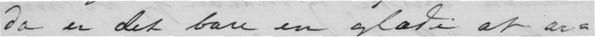da er det bare en glæde at ar-
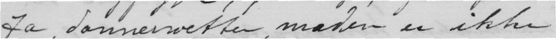Ja, donnerwetter, maden er ikke
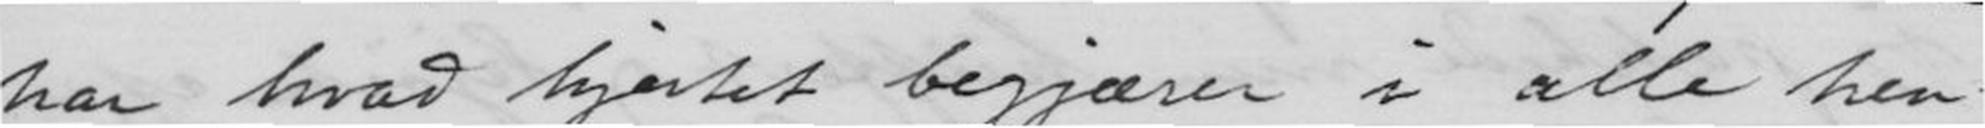har hvad hjertet begjærer i alle hen-
